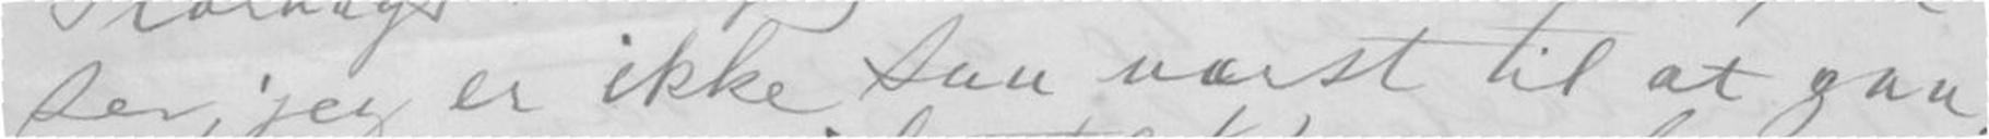ser; jeg er ikke saa værst til at gaa.
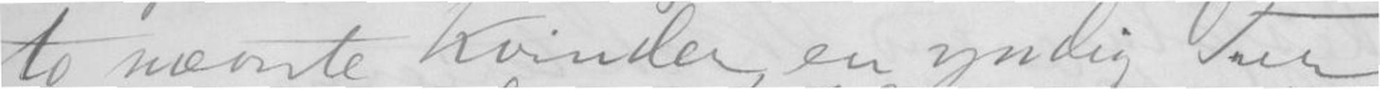to nævnte Kvinder, en yndig Tur.
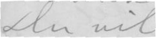Du vil.
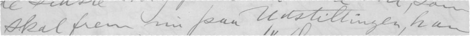skal frem nu paa Udstillingen, han
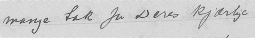mange tak for Deres kjærlige
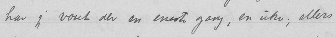har jeg været der en eneste gang, en uke; ellers
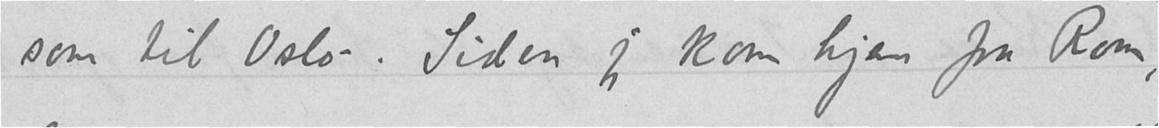som til Oslo –. Siden jeg kom hjem fra Rom,
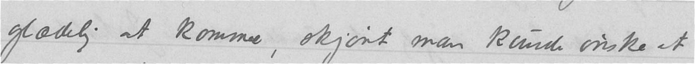glædelig at komme, skjønt man kunde ønske at
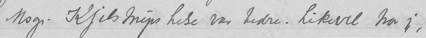Msgr. Kjelstrups helse var bedre. Likevel tror jeg,
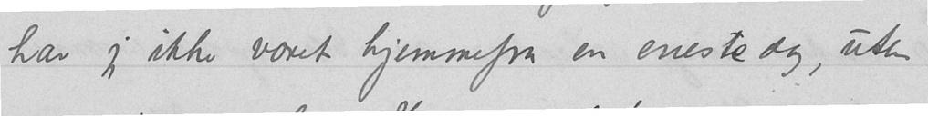har jeg ikke været hjemmefra en eneste dag, uten
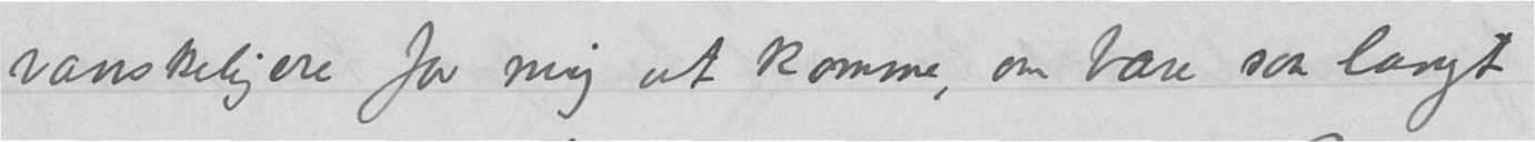vanskeligere for mig at komme, om bare saa langt
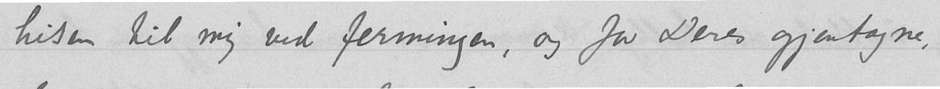hilsen til mig ved fermingen, og for Deres gjentagne,
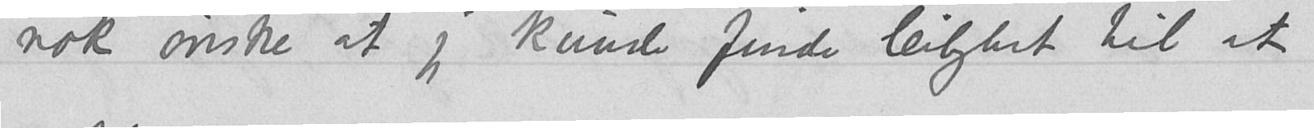nok ønske at jeg kunde finde leilighet til at
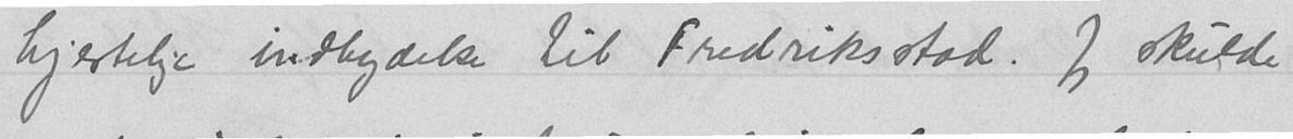hjertelige indbydelse til Fredriksstad. Jeg skulde
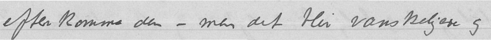efterkomme den – men det blir vanskeligere og
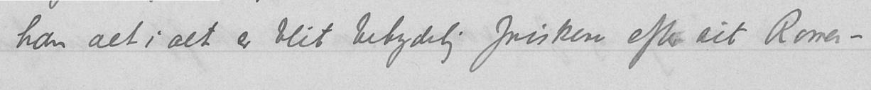han alt i alt er blit betydelig friskere efter sit Roma-
